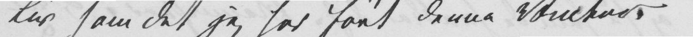Liv som det jeg har ført denne Vinter.
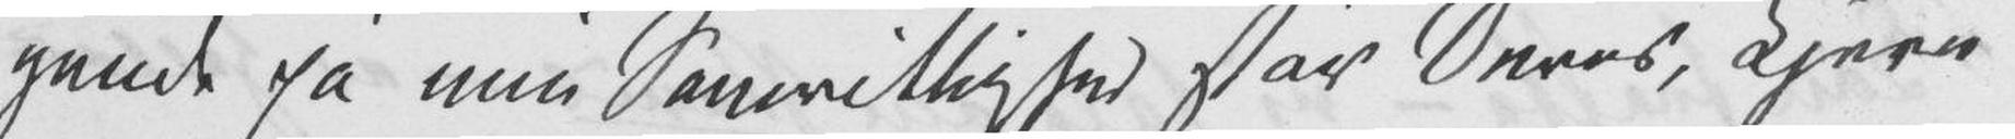gende på min Samvittighed står Deres, kjere
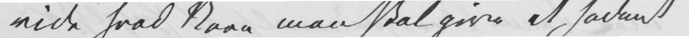vide hvad Navn man skal give et sådant
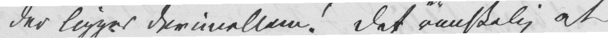der ligger derimellem! Det er vanskelig at
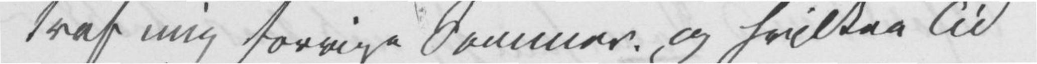traf mig forrige Sommer, og hvilken Tid
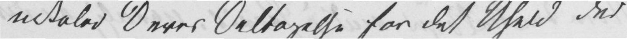udtaled Deres Deltagelse for det Uheld der
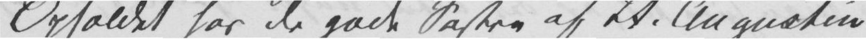Opholdet hos de gode Søstre af St. Augustin
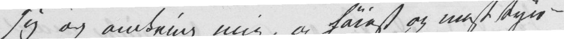sig op omkring mig, og høiest og mest tyn¬
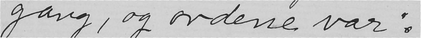gang, og ordene var:
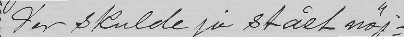Der skulde jo stået nøj-
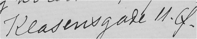Klasensgade 11. Ø.
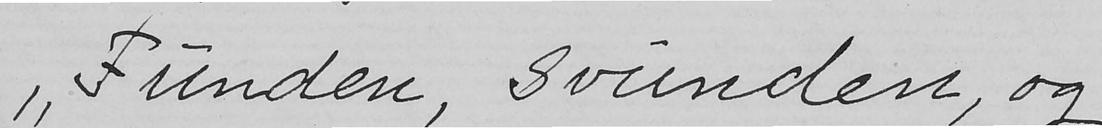"Funden, svunden, og
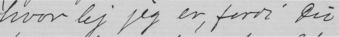hvor lej jeg er, fordi Du
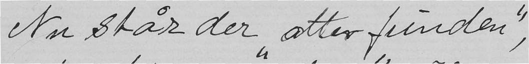Nu står der "atter funden",
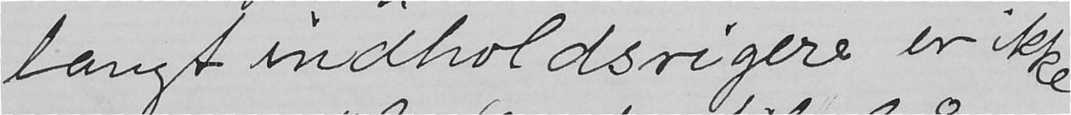langt indholdsrigere er ikke
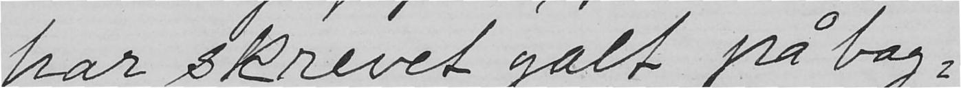har skrevet galt på bag-
